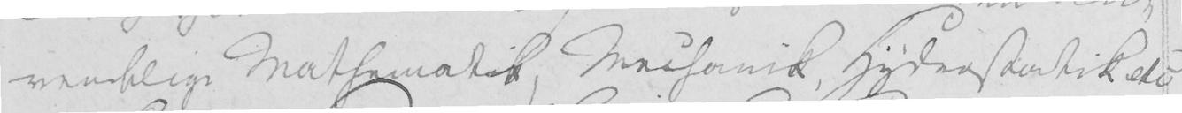vendelige Mathematik, Mechanik, Hydrostatik etc
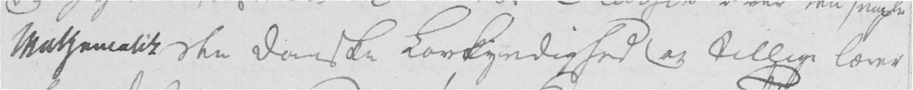Mathematik den danske Lovkyndighed og tillige lærer
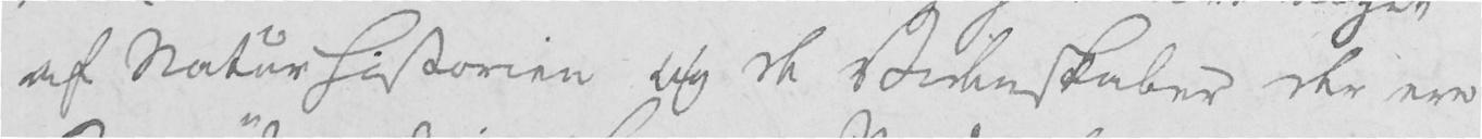af Naturhistorien og de Videnskaber der ere
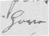have
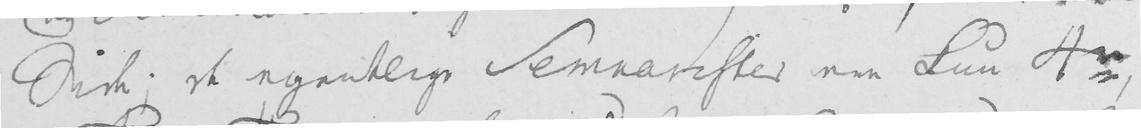Side; de egentlige Semnarister er kun 4re,
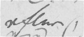efter
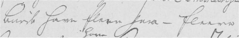burde have flere hesa - fleere
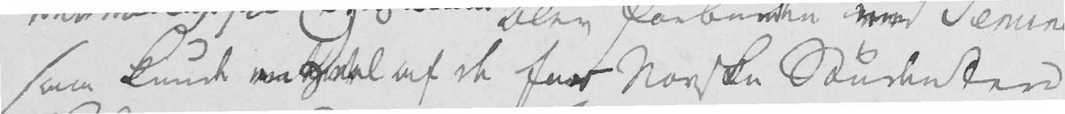saa kunde en Deel af de faa Norske Studenter
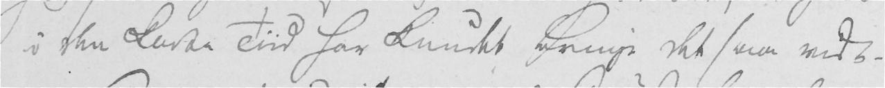i den Laste Tiid har kundet bruge det saa vidt -
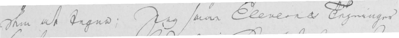dem at tegne; Jeg saae Elevernes Tegninger
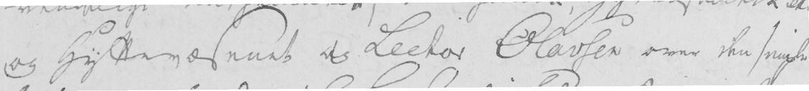og Hyttevæsenet og Lector Olavsen over den singte
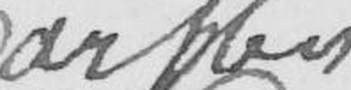arsten
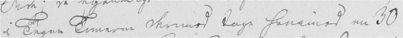i Tegne Timerne derimod tage henimod en 30
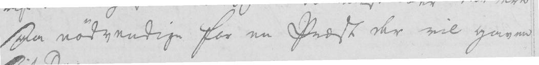saa nødvendige for en Præst der vil gavne
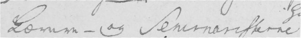Lærere - og Seminaristerne
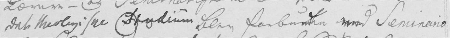det theologiske Studium blev forbudte ved Seminario
